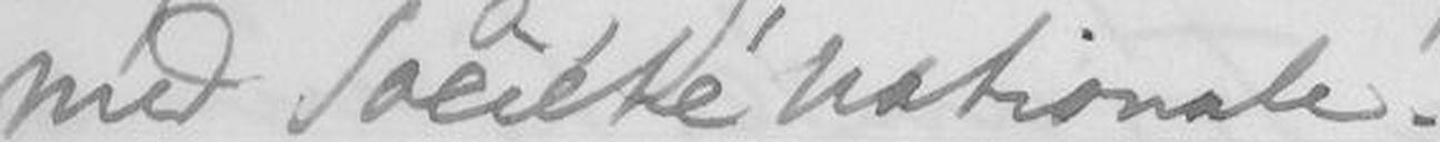med Soeiété Nationale.
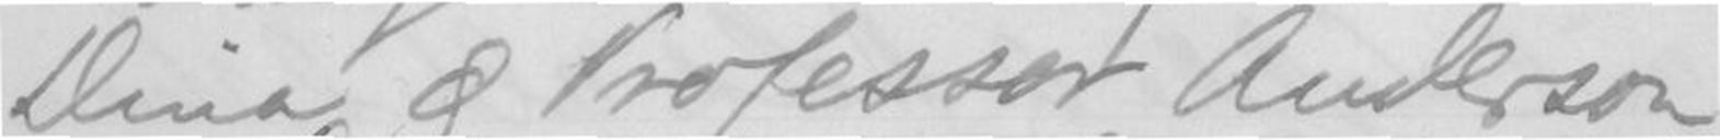Dina og Professor Anderson.
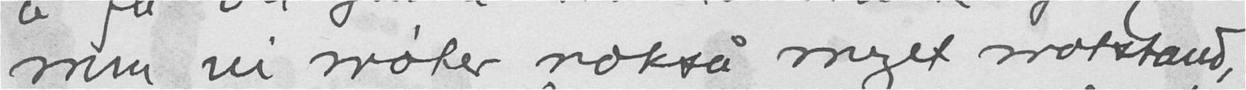men vi møter nokså meget motstand,
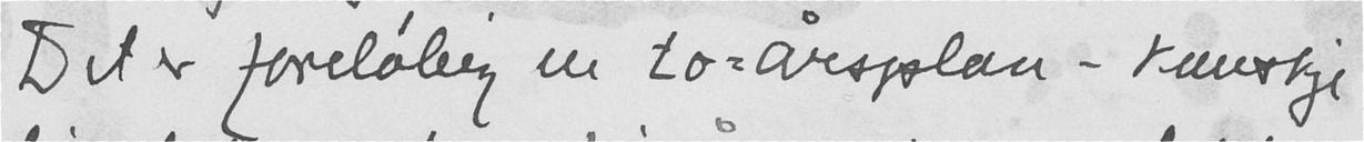Det er foreløbig en to-årsplan - plutselig
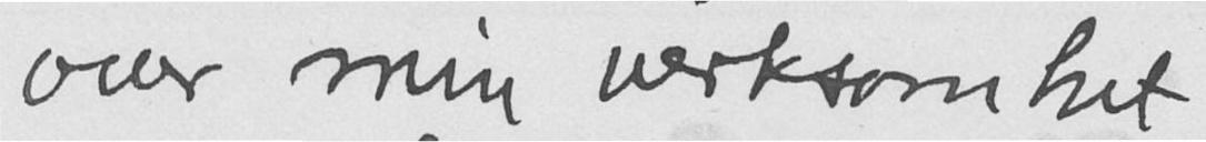over min virksomhet
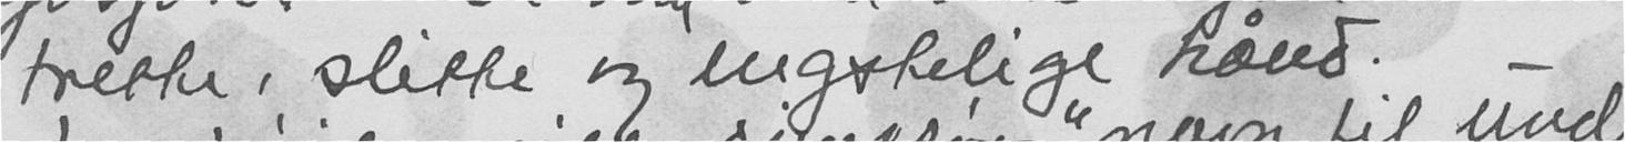treshe, slitte og lngstelige hånd. -
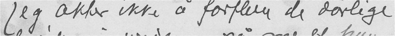Jeg akter ikke å forflere de dårlige
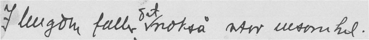I lengden faller det nokså stor insomhel.
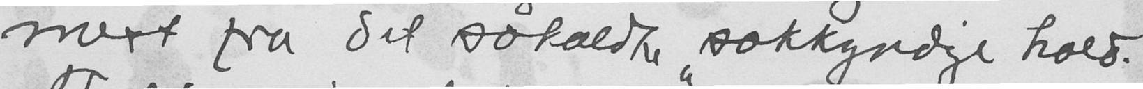mest fra det såkaldt "sakkyndige hold."
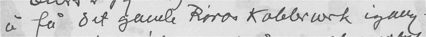å få det gamle Røros Kobberverk igang,
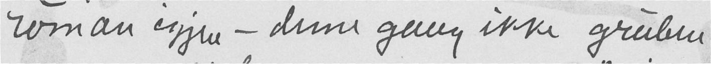roman igjen - denne gang ikke gruben
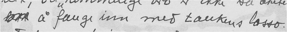lett å fange inn med tankens lasso.
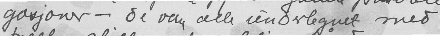gasjoner - De var alle undertegnet med
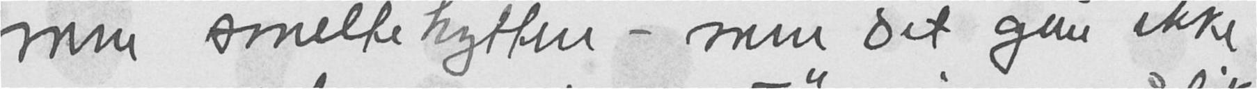men smeltehytten - men det går ikke
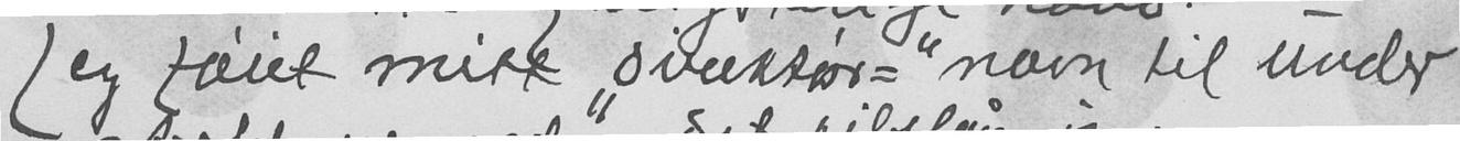Jeg føiet mitt "Svektor-"navn til under
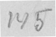145
